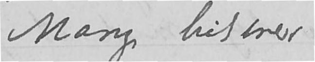Mange hilsener
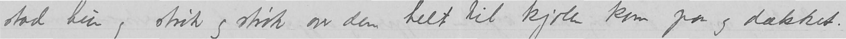stod hun og strøk og strøk over dem helt til kjolen kom paa og dækket.
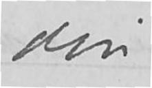din
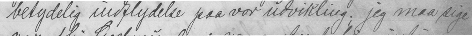betydelig indflydelse paa vor udvikling; jeg maa sige
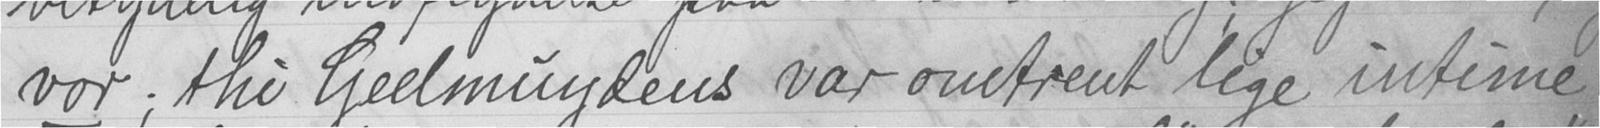vor; thi Geelmuydens var omtrent lige intime
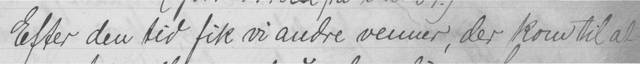Efter den tid fik vi andre venner, der kom til at
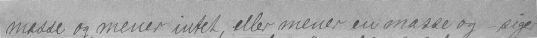masse og mener intet, eller mener en masse og - siger
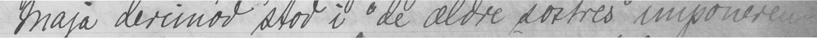Maja derimod stod i "de ældre søstres imponeren-
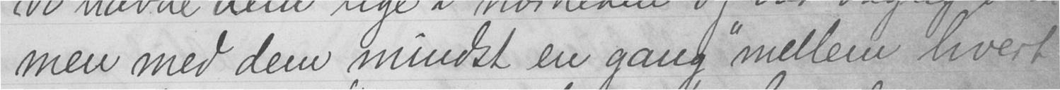men med dem mindst en gang "mellem hvert
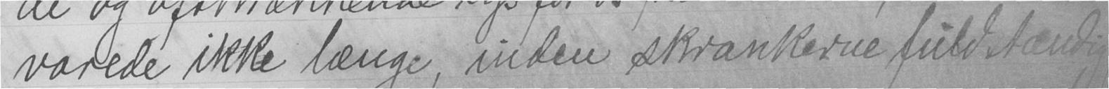varede ikke længe, inden skrankerne fuldstændig
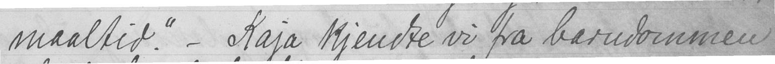maaltid". - Kaja kjendte vi fra barndommen
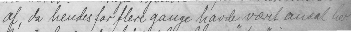af, da hendes far flere gange havde været ansat her.
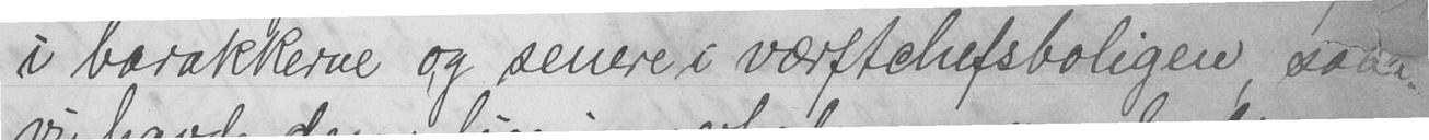i barakkerne og senere i værftchefsboligen, saa
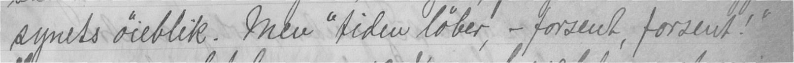synets øieblik. Men "tiden løber, - forsent, forsent!"
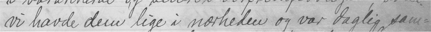vi havde dem lige i nærheden og var daglig sam-
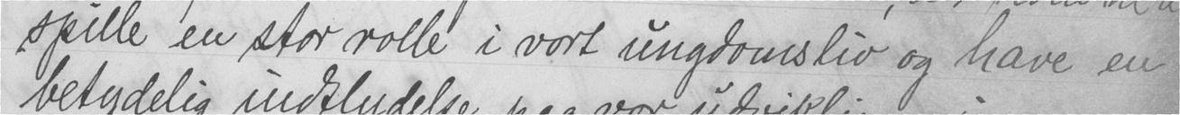spille en stor rolle i vort ungdomsliv og have en
